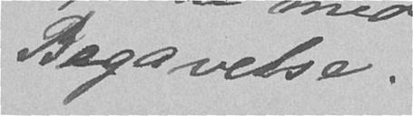Bagavelse.
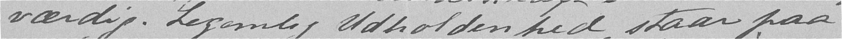værdig. Legemlig Udholdenhed staar paa
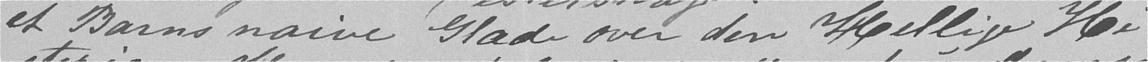et Barns naive Glæde over den Hellige Hi-
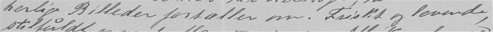herlige Billeder forteller om. Friskt og levende,
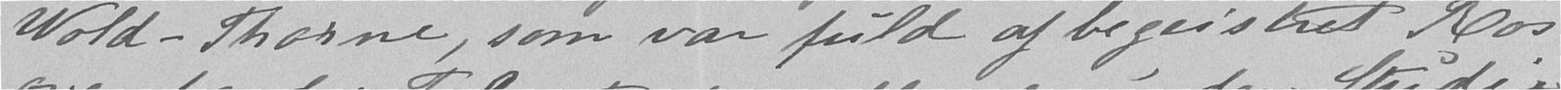Wold. Thorne, som var fuld af begeistret Ros
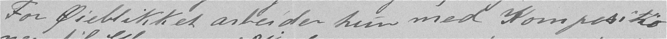For Øieblikket arbeider hun med Kompositio
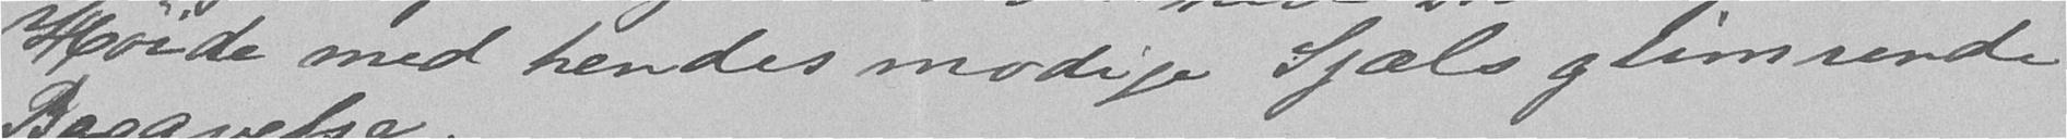Høide med hendes modige Sjæls glimrende
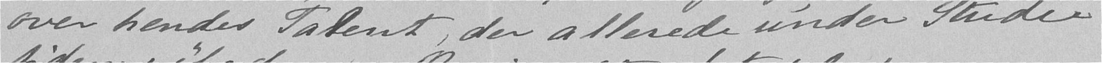over hendes Tatent, der allerede under Studie
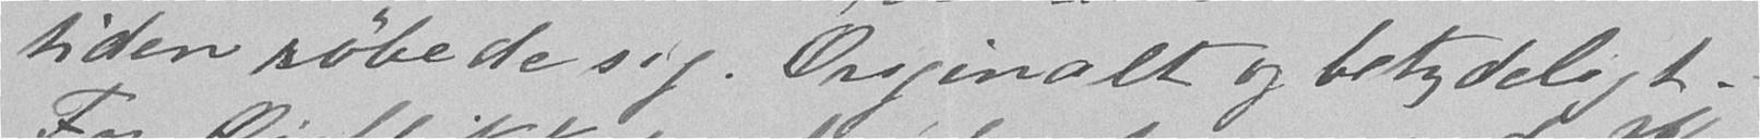tiden røbede sig. Origenalt og betydeligt.-
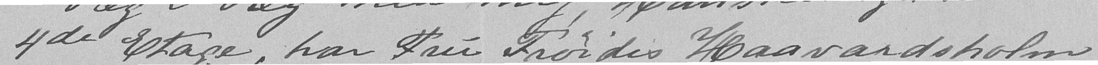4de Etage, har Fru Frøidos Haavardsholm
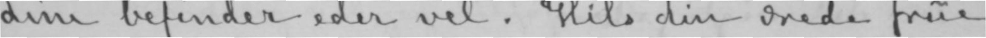dine befinder eder vel. Hils din ærede frue
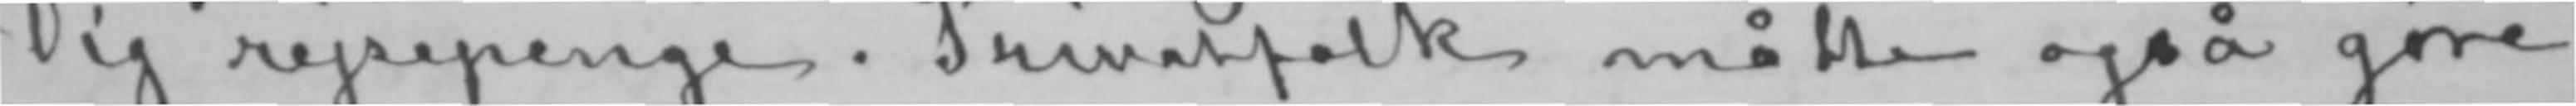Dig rejsepenge. Privatfolk måtte også gøre
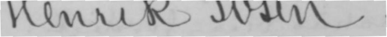Henrik Ibsen.
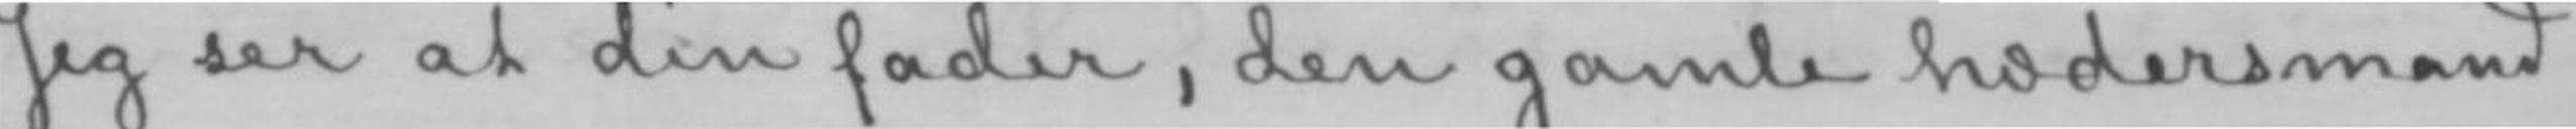Jeg ser at din fader, den gamle hædersmand
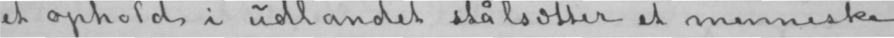et ophold i udlandet stålsætter et menneske
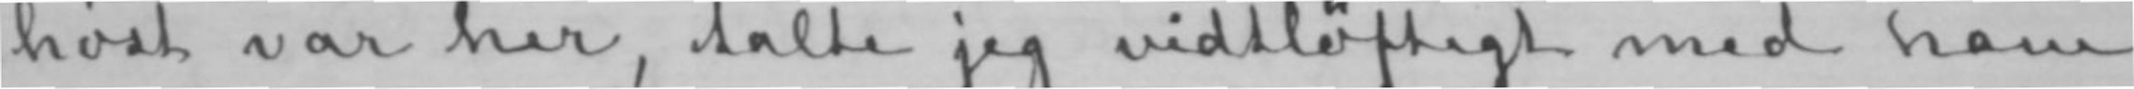høst var her, talte jeg vidtløftigt med ham
